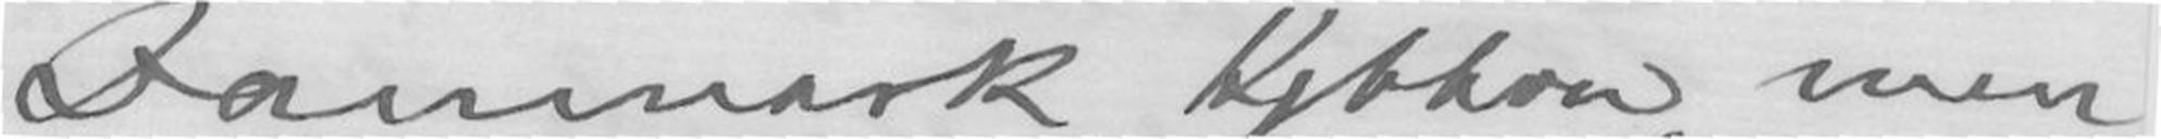Danmark, kjbhvn, men
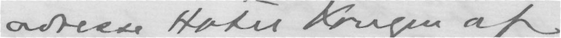adresse Hotel Kongen af
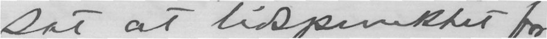sat at Tidspunktet for
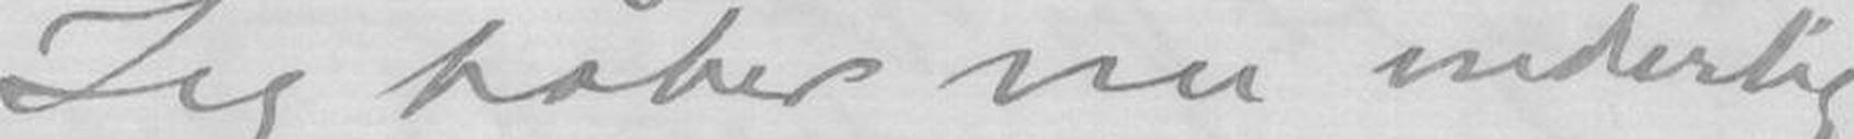Jeg håber nu inderlig
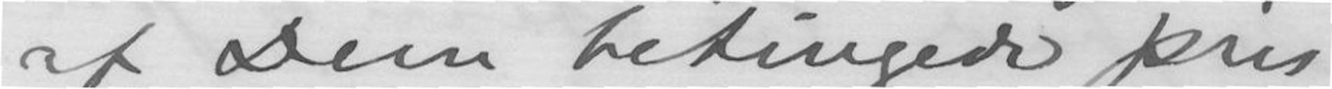af Dem betingede pris
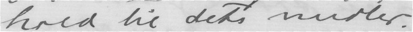hold til dets midler.
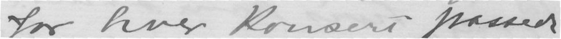for hver konsert passede
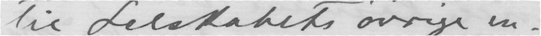til Selskabets øvrige en-
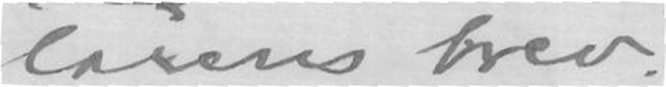tærens brev.
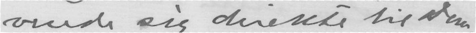vende sig direkte til Dem,
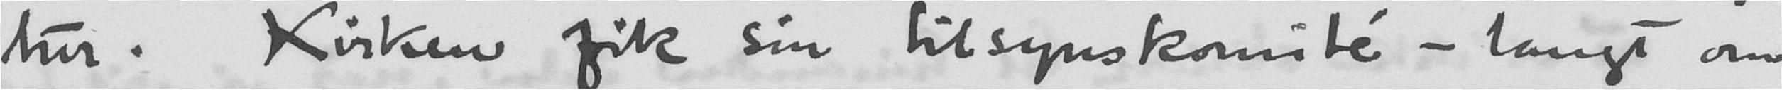tur. Kirken fik sin tilsynskomité - langt om
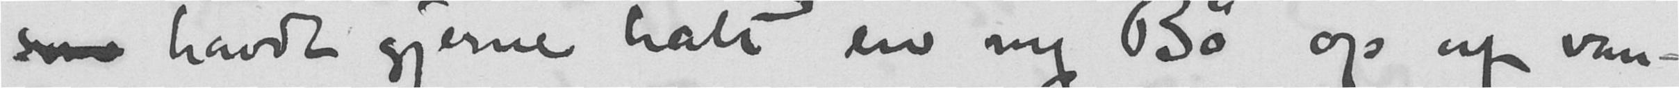som havde gjerne halt en ny Bø op af van-
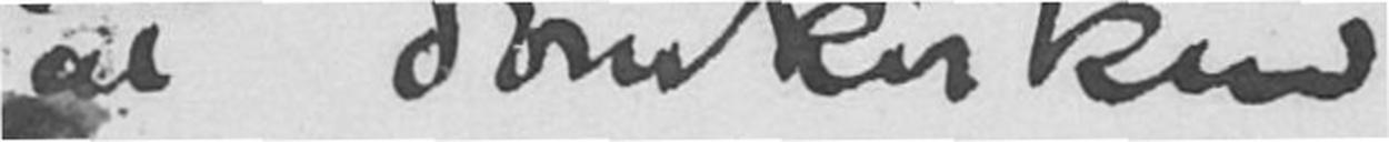at domkirken
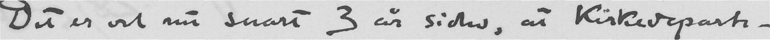Det er vel nu snart 3 år siden, at Kirkedeparte-
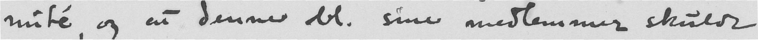mité, og at denne bl. sine medlemmer skulde
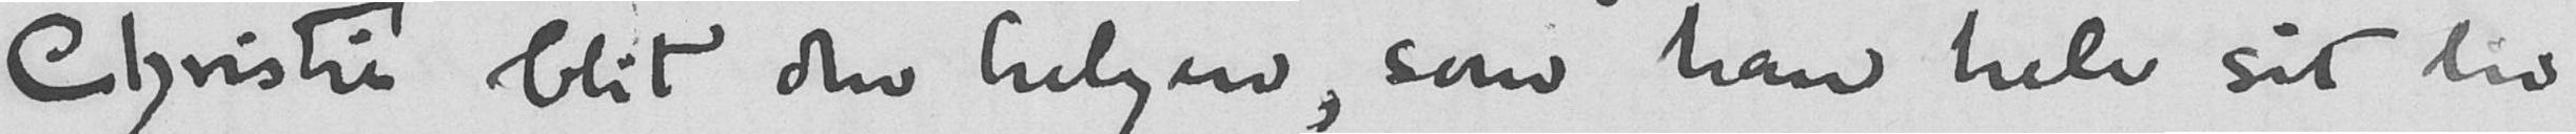Christie blit den helgen, som han hele sit liv
